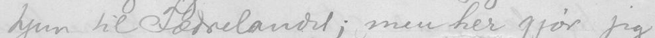hjem til Fædrelandet; men her gjør jeg
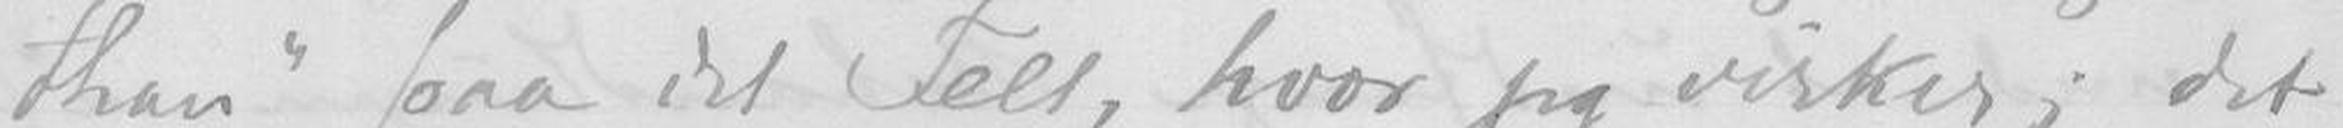than paa det Felt, hvor jeg virker; det
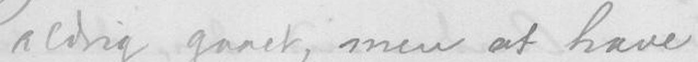aldrig gaaet, men at have
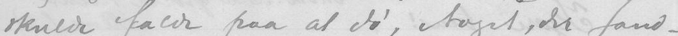skulde falde paa at dø, Noget, der sand-
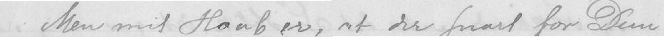Men mit Haab er, at der snart for Dem
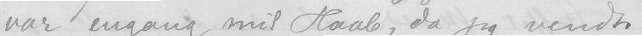var engang mit Haaab, da jeg vendte
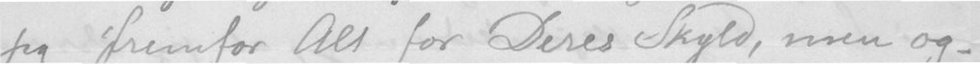jeg fremfor Alt for Deres Skyld, men og-
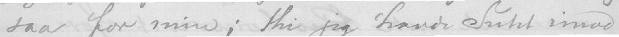saa for min; thi jeg havde Intet imod
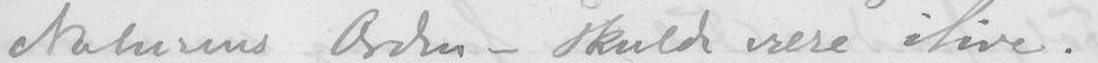Naturens Orden - skulde være ilive.
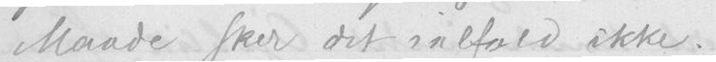Maade sker det ialfald ikke.
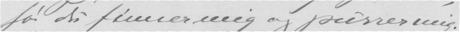før du finner mig og purrer mig.
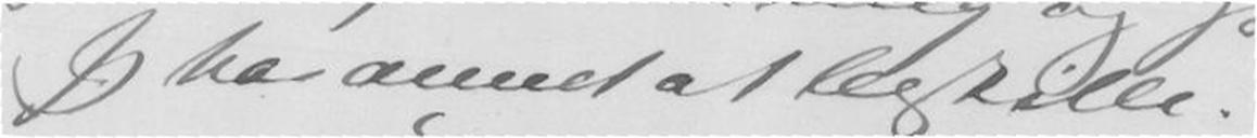Jeg har annet at bestille.
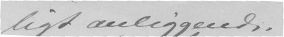ligt anliggende.
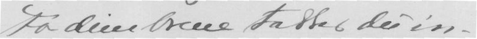For dine breve takkes du in-
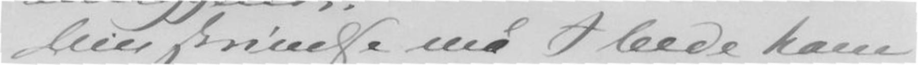Min skrivelse må I bede ham
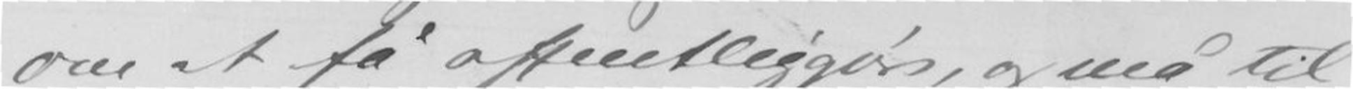om at få offentliggøre, og må til
